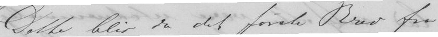Dette blir da det første Brev fra
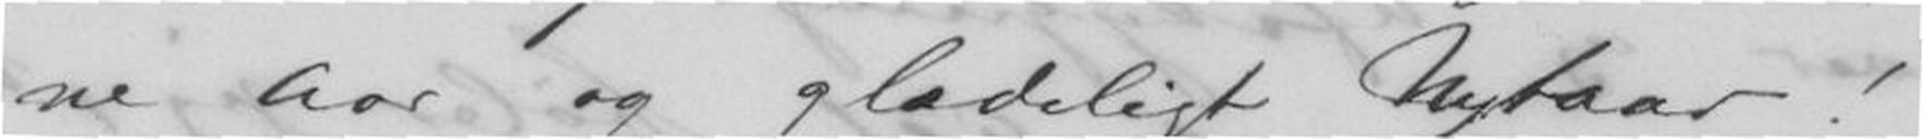ne Aar og glædeligt Nytaar! -
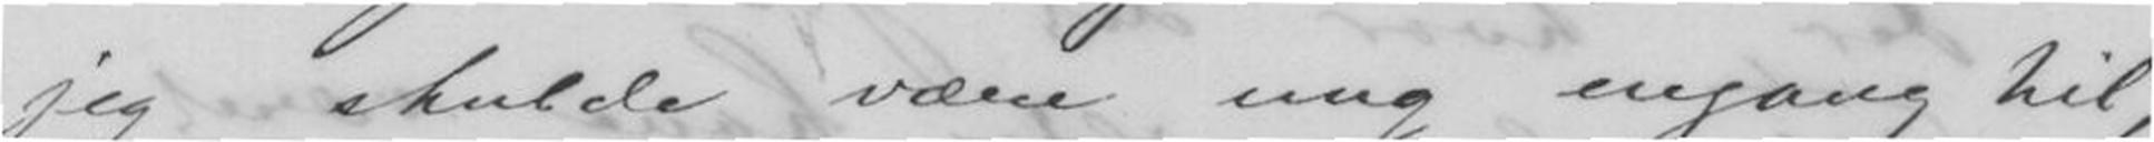jeg skulde være ung engang til,
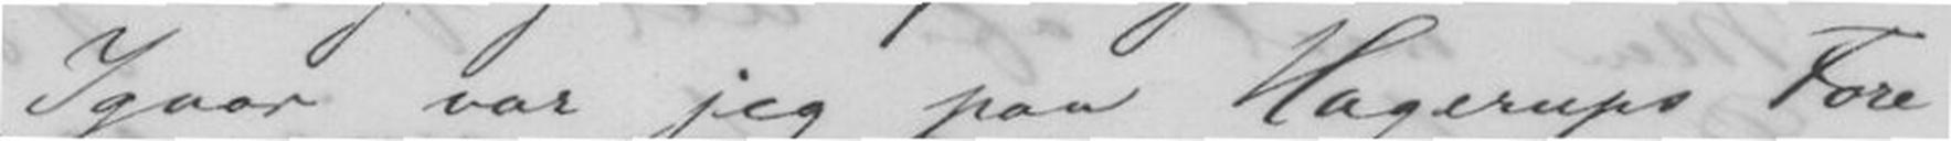Igaar var jeg paa Hagerups Fore-
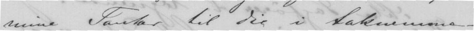mine Tanker til dig i taknemme-
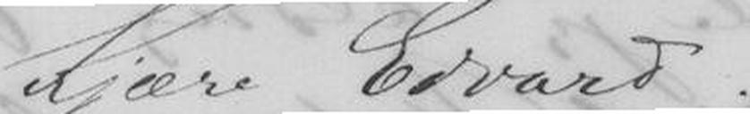Kjære Edvard!
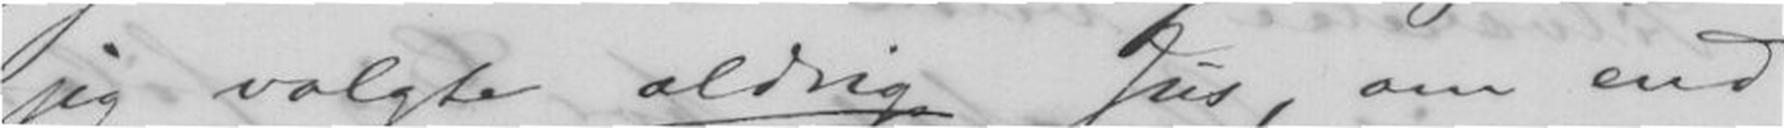jeg valgte aldrig Jus, om end
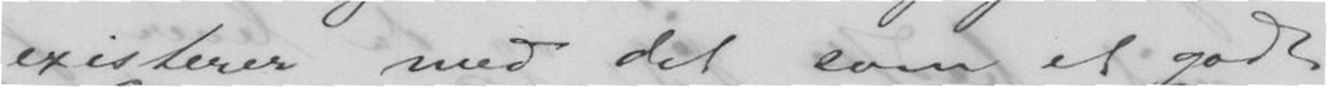existerer med det som et godt
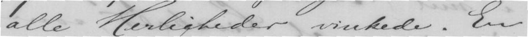alle Herligheder vinkede. En
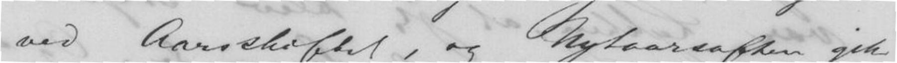ved Aarskiftet, og Nytaarsaften gik
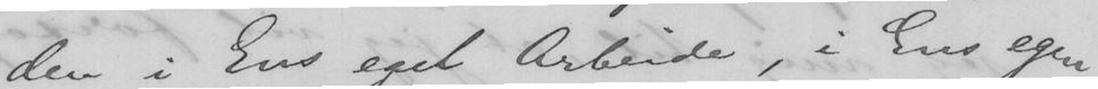den i Ens eget Arbeide, i Ens egen
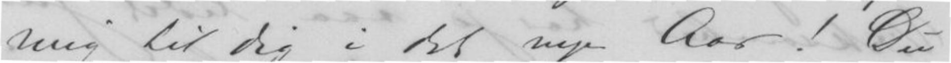mig til dig i det nye Aar! Du
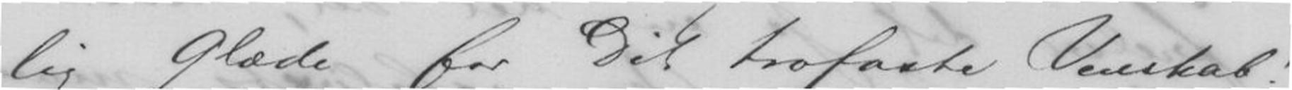lig Glæde for Dit trofaste Venskab!
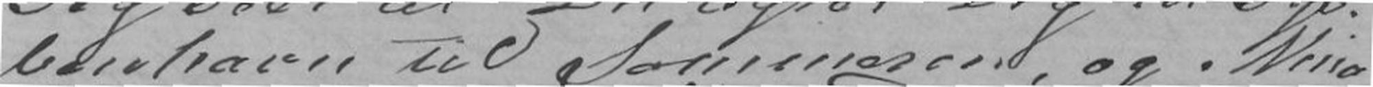benhavn til Sommeren, og Nina
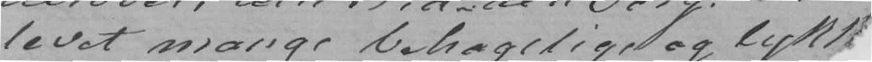levet mange behagelige og Lykk
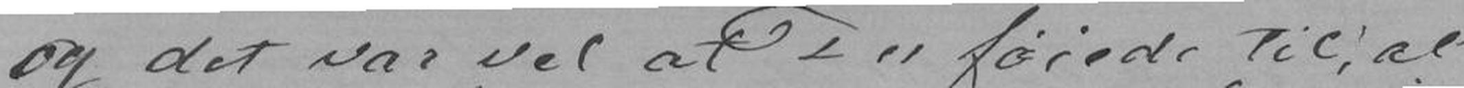og det var vel at Vu føiede til, at
